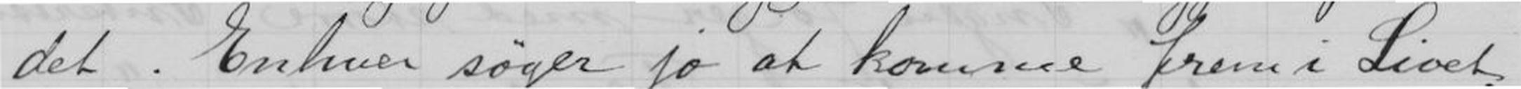det. Enhver søger jo at komme frem i Livet,
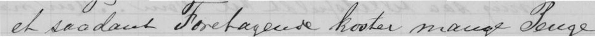et saadant Foretagende koster mange Penge,
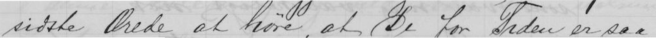sidste Ærede at høre, at De for Tiden er saa
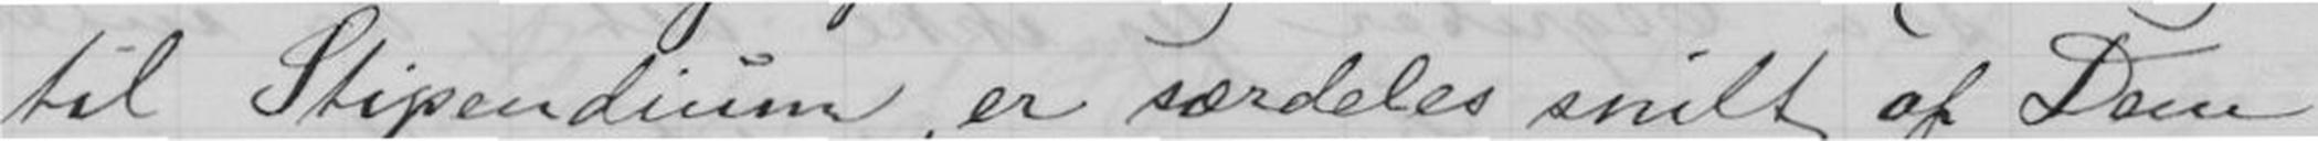til Stipemdium, er særdeles snilt af Dem
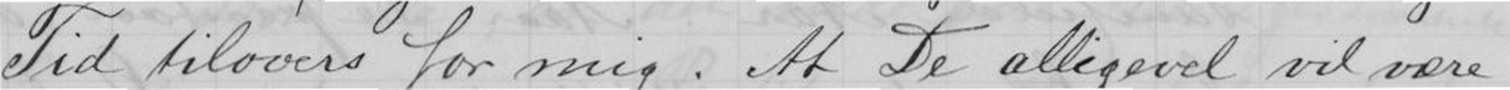Tid tilovers for mig. At De alligevel vil være
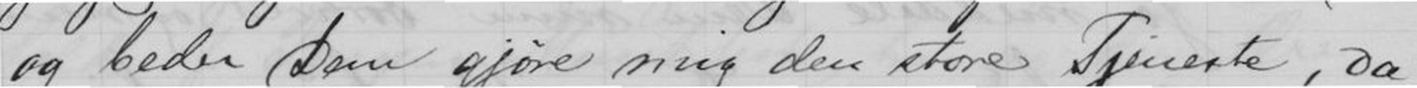og beder Dem gjøre mig den store Tjeneste, da
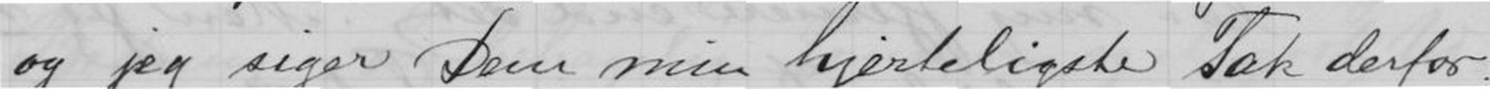og jeg siger Dem min hjerteligste Tak derfor
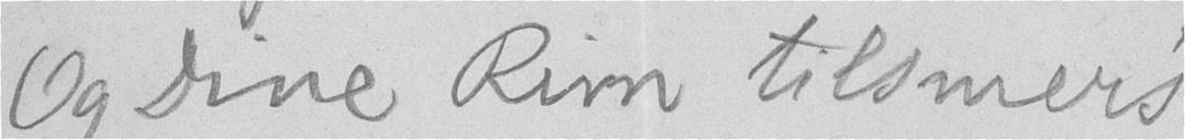Og Dine Rim tilsmers
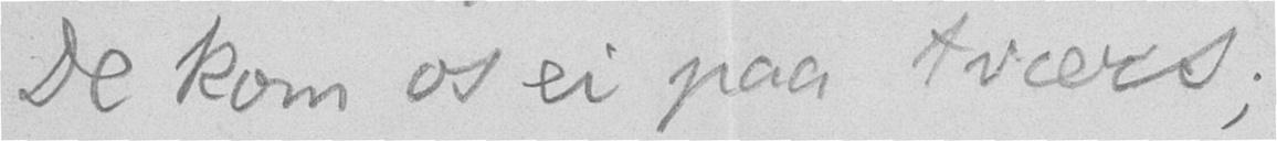De kom os ei paa tværs;
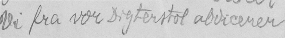Vi fra vor Digterstol abdicerer.
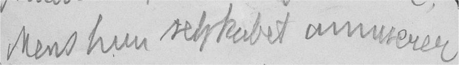Mens hun selskabet annulerer,
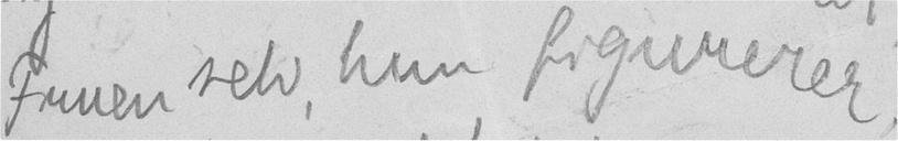Fruen selv, hun figurerer
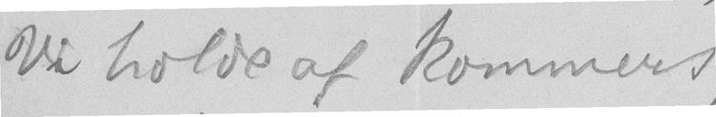Vi holde af kommers,
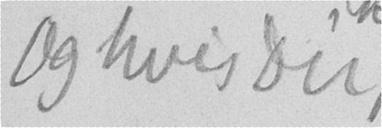Og hvis Du
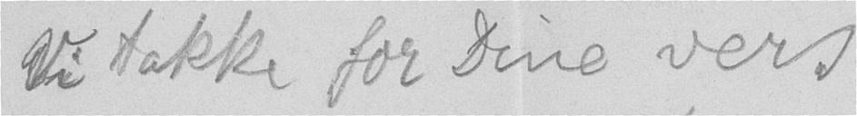Vi takke for Dine vers
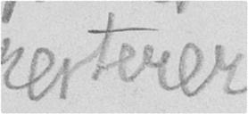rerterer,
